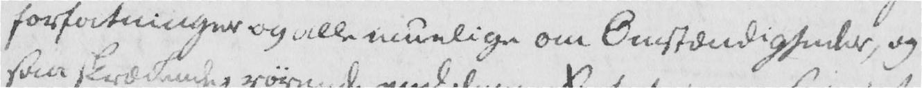forfatninger og alle muelige om Omstændigheder, og
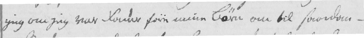jeg om jeg var Fader føre mine Børn om til saadan-
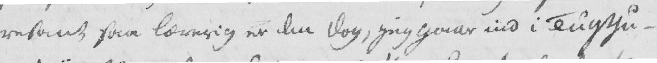resant saa lærerig er den dog, jeg gaar ind i Tugthu-
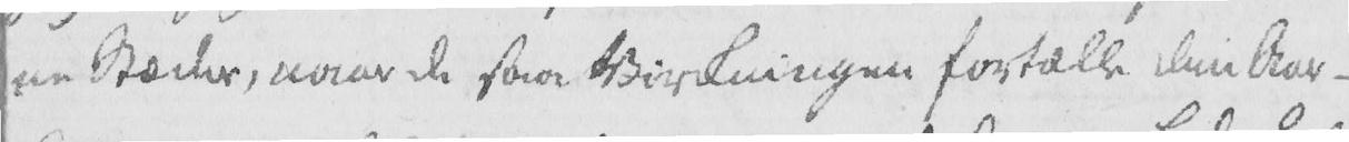ne Stæder, naar de saa Virkningen fortælle dem Aar-
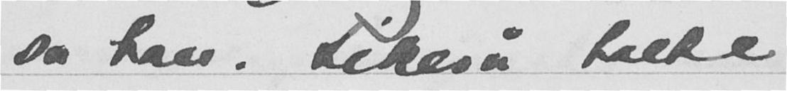sa han. Likeså talte
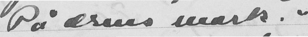"På ærens mark."
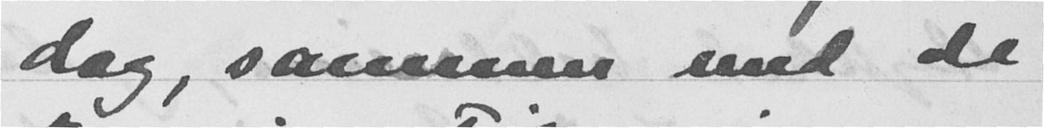dag, sammen med de
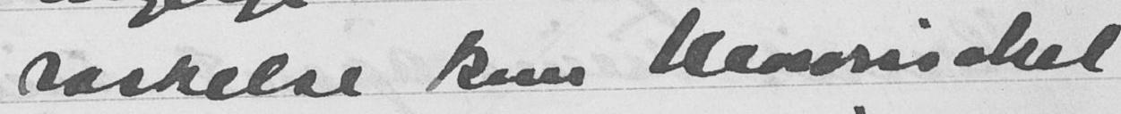raskelse kom Mowinckel
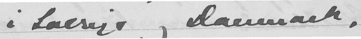i Sverige og Danmark,
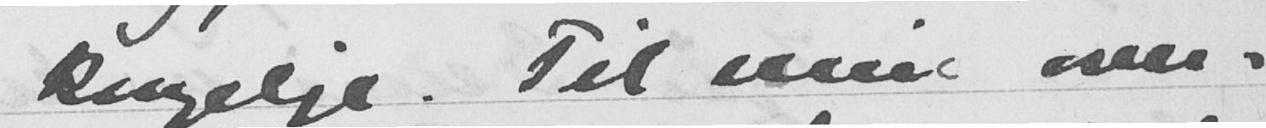kongelige. Til min over-
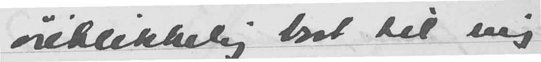øieblikkelig bort til mig
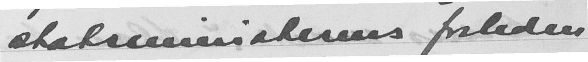statsministerens forleden
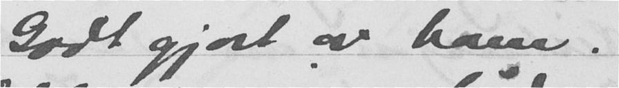Godt gjort av ham.
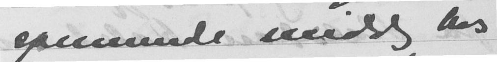spennende middag hos
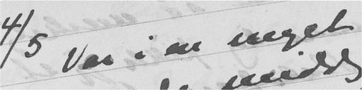4/5 Var i en meget
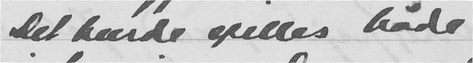Det burde spilles både
